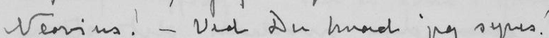Nevius!- Ved Du hvad jeg synes?
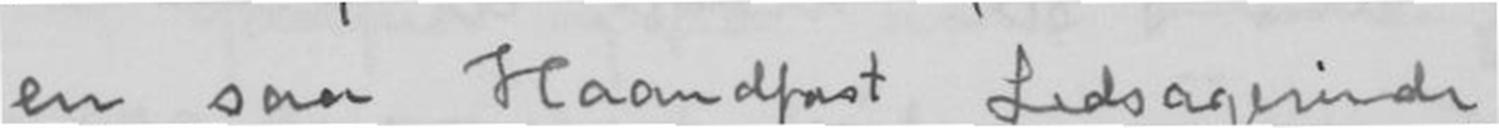en saa Haandfast Ledsagerinde
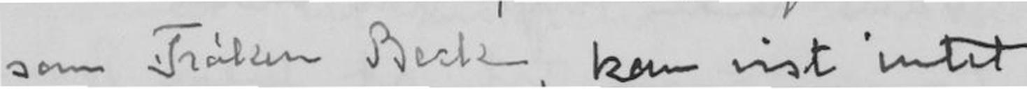som Frøken Beck, kan vist intet
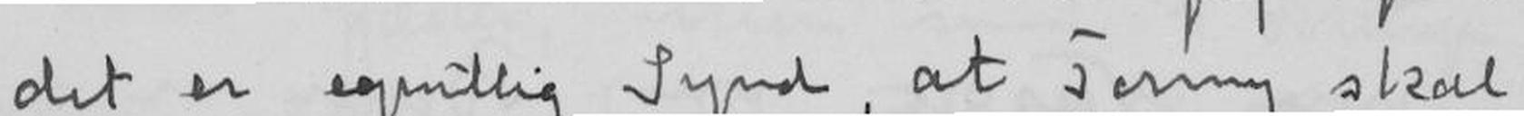det er egentlig Synd, at Tonny skal
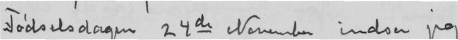Fødselsdagen 24de November indser jeg
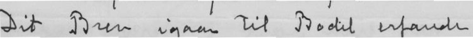Dit Brev igaar til Bodil erfarede
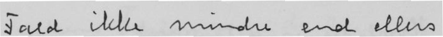fald ikke mindre end ellers
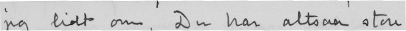jeg lidt om, Du har altsaa store
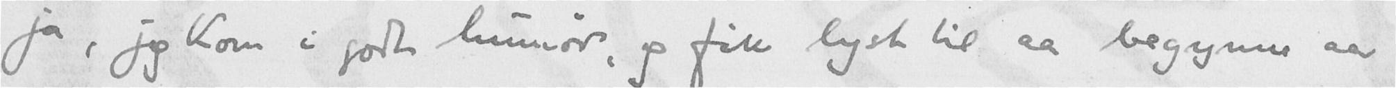Ja, jeg kom i godt humør og fik lyst til aa begynne aa
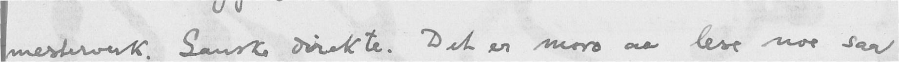mesterverk. Ganske direkte. Det er morro å lese noe saa
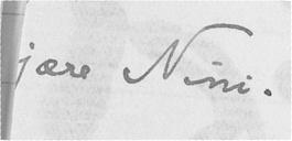Kjære Nini.
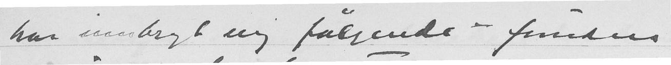har innbragt mig følgende firters
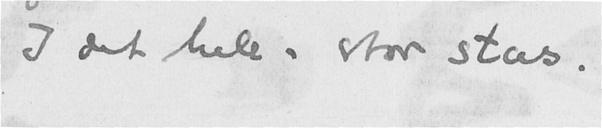I det hele, stor stas.
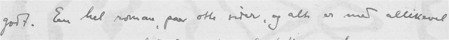godt. En hel roman paa otte bider, og alt er med allikevel
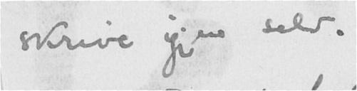skrive igjen selv.
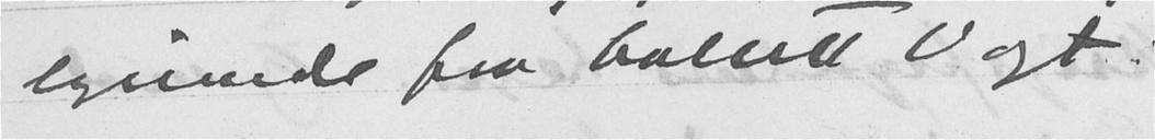lgnende fra Collett Vagt.
